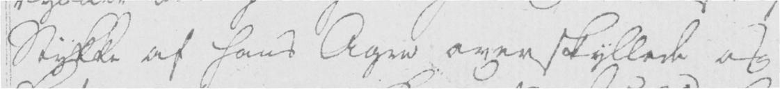Stykke af hans Ager overskyllede og
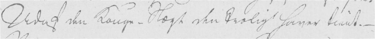Udaf den Konge-Slægt den trolig haver tient. -
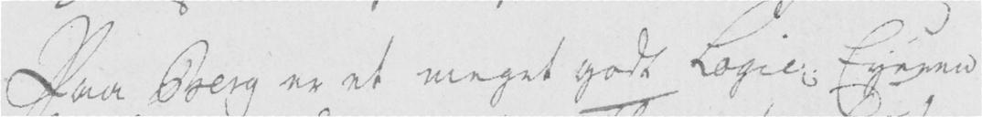Paa Berg er et meget godt Logie; Eyeren
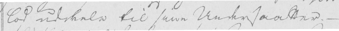lod uddeele til sine Undersaatter. -
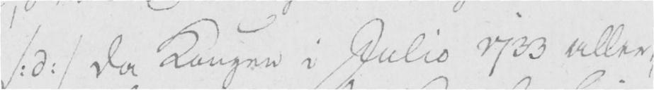/: d :/ da Kongen i Julio 1733 aller-
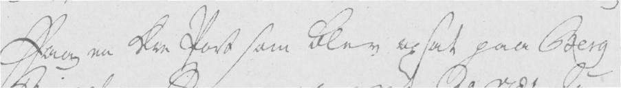Paa en Ære Port som blev opsat paa Berg
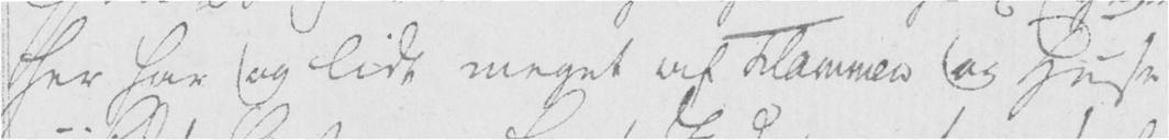her har og lidt meget at Flommen og Huset
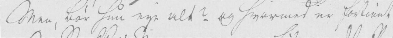Men, bør hun eye alt? og hvormed er fortient
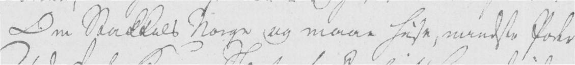Om Stakkels Norge og maae huse, mindste Pode
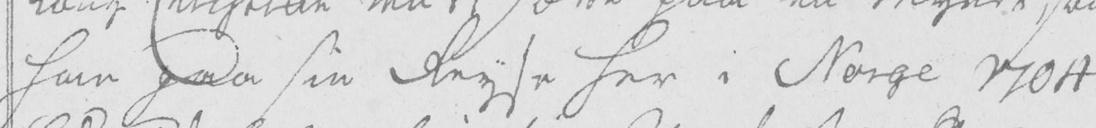han paa sin Reyse her i Norge 1704
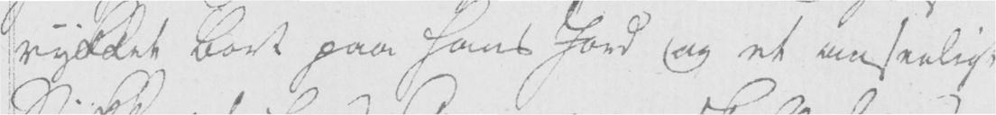rykket bort paa hans Jord og et anseeligt
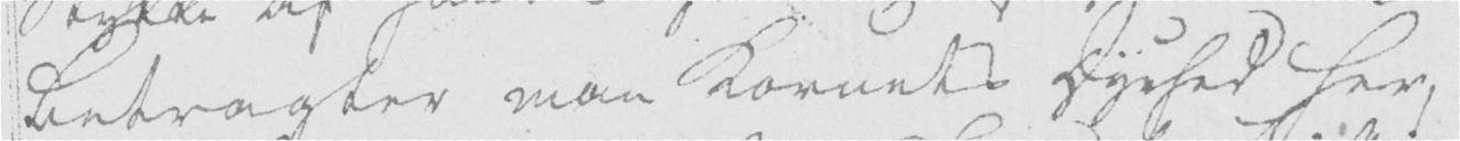betragter man Kornets Dyrhed her,
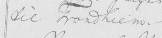til Trondhiem. -
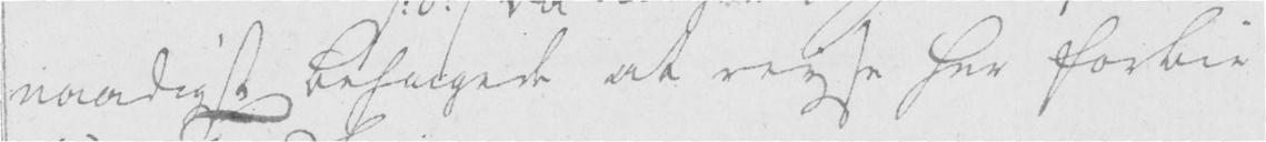naadigst besørgede at reyse her forbie
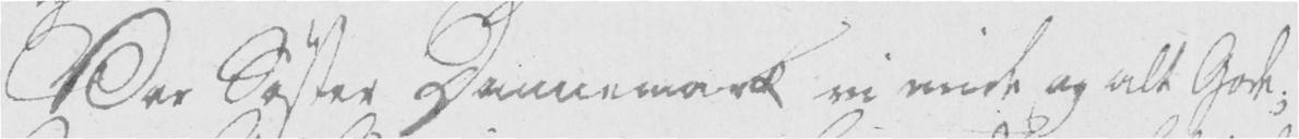Vor Søster Dannemark vi unde og alt Godt;
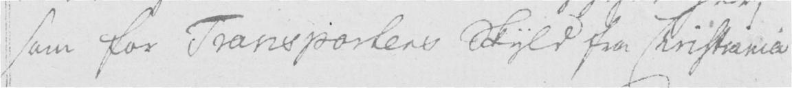som for Transportens skyld fra Christiania
